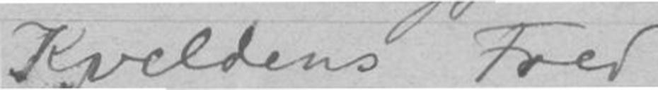Kveldens Fred
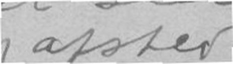afsted
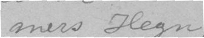mers Hegn,
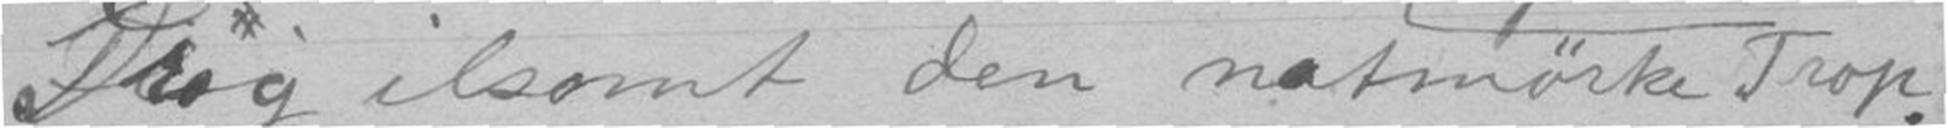Drog ilsomt den natmørke Trop.
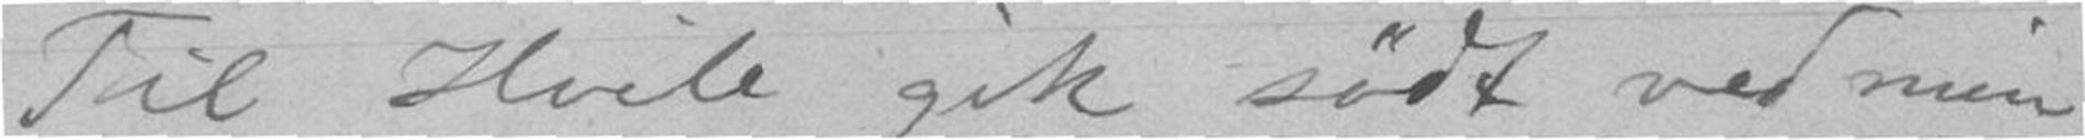Til Hvile gik sødt ved min
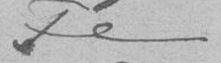Fe
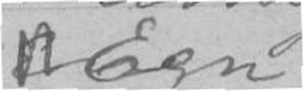Egn
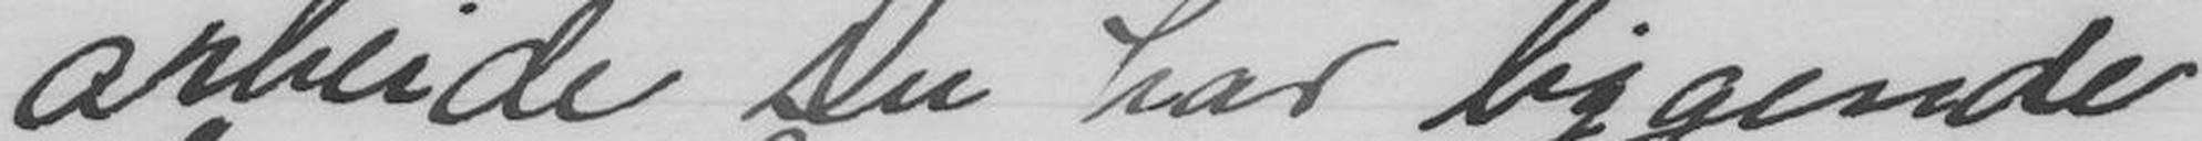arbeide Du har ligende
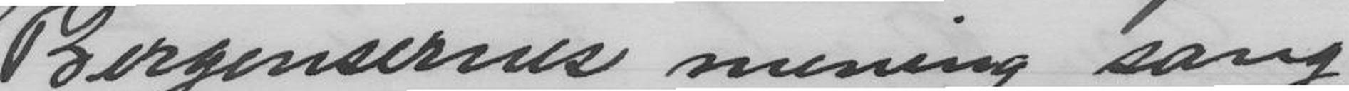Bergensernes mening sang
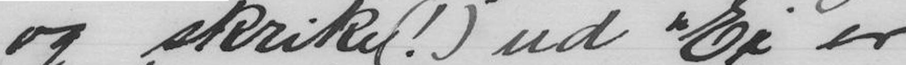og skrike(!) ud "Ei er
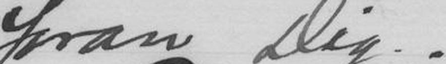foran Dig.
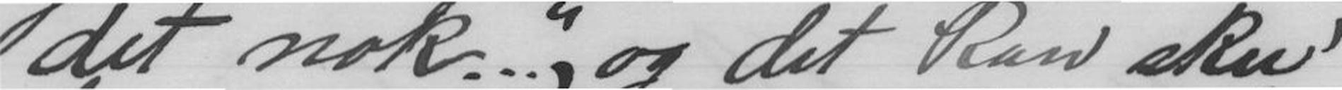det nok"..., og det kan sku'
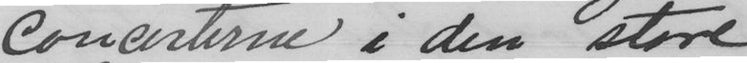Concerterne i den store
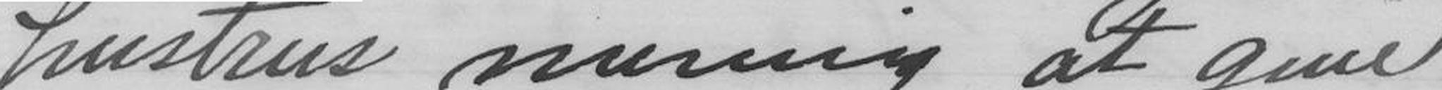hustrus mening at give
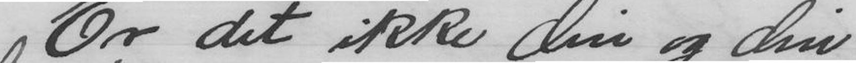Er det ikke din og din
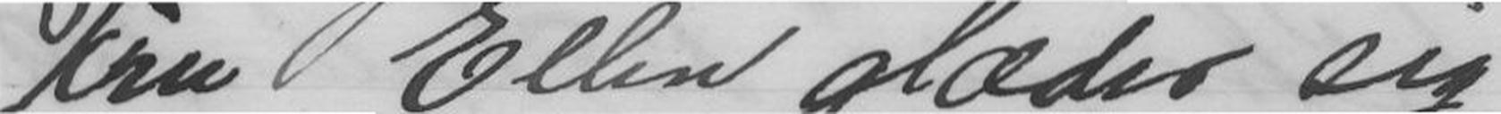Fru Ellen glæder sig
